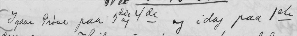Igaar Prøve paa 3die og 4de og idag paa 1ste
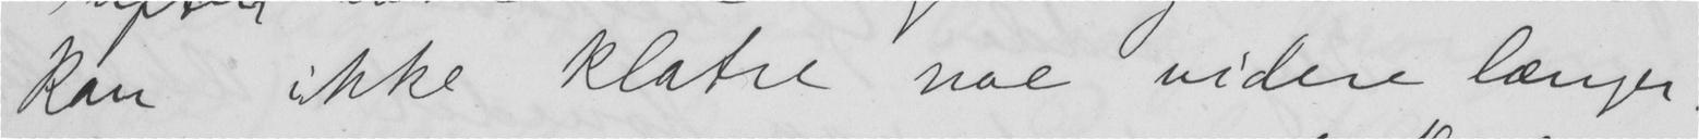kan ikke klatre noe videre længer.
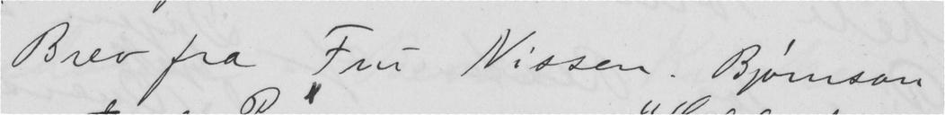Brev fra Fru Nissen. Bjørnson
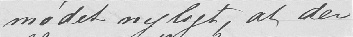mødet nyligt, at der
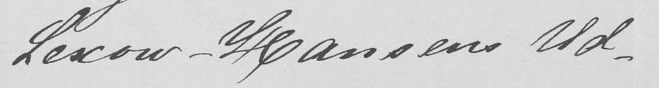Lexow-Hansens Ud-
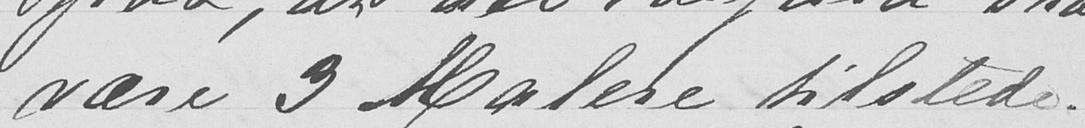være 3 Malere tilstede.
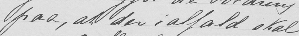paa, at der ialfald skal
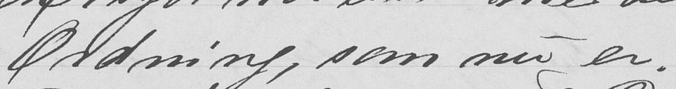Ordning, som nu er.
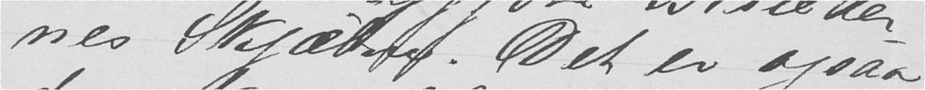nes Skjæbne. Det er ogsaa
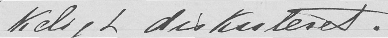keligt diskuteret.
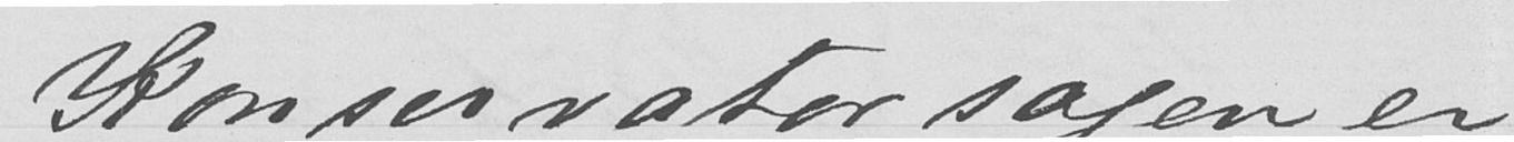Konservatorsagen er
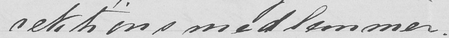rektionsmedlemmer.
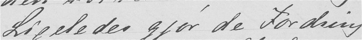Ligeledes gjør de Fordring
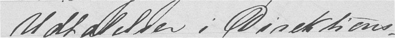Udtalelser i Direktions-
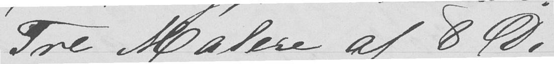Tre Malere af 8 Di-
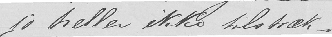jo heller ikke tilstræk-
